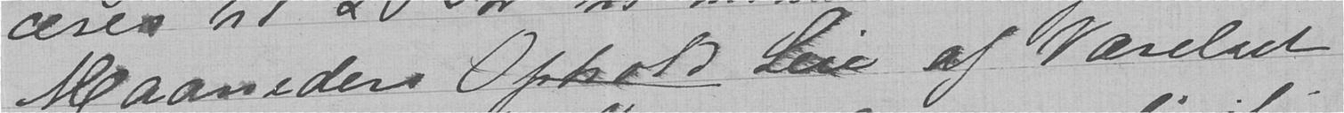Maaneders Ophold Leie af Værelset
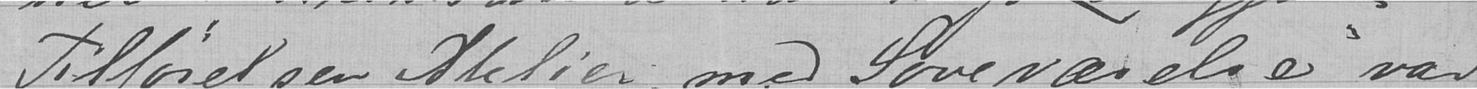Tilføielsen Atelier, med Soveværelse var
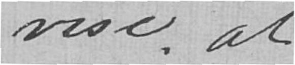vise at
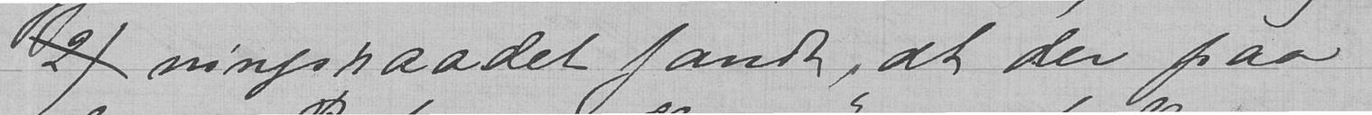2) ningsraadet fandt, at der paa
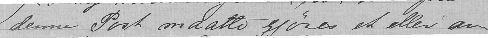denne Post maatte gjøres et eller an-
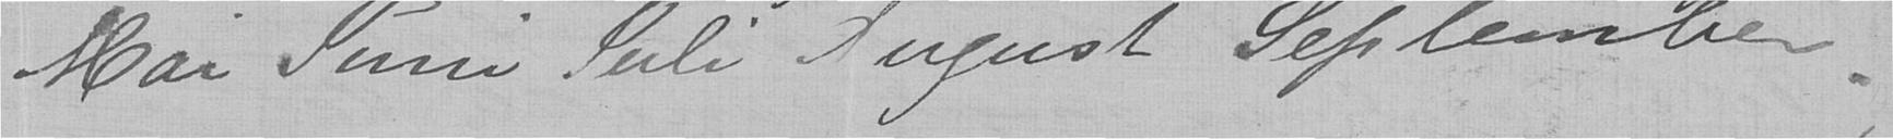Mai Juni Juli August September.
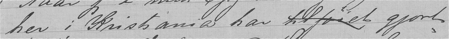her i Kristiania har tilføiet gjort
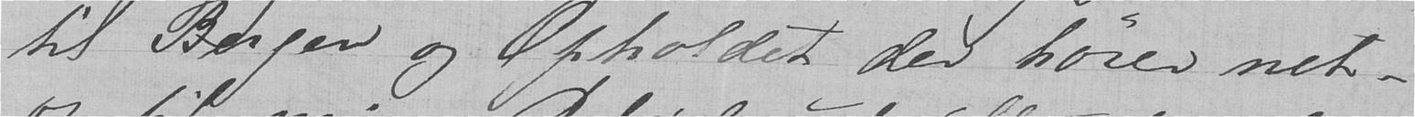til Bergen og Opholdet der hører net-
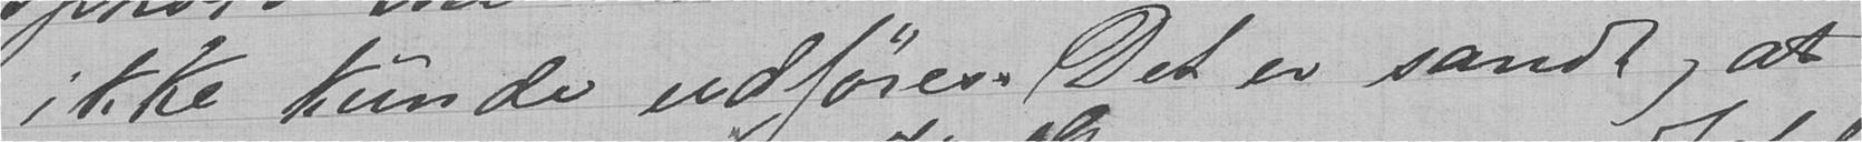ikke kunde udføres. Det er sandt, at
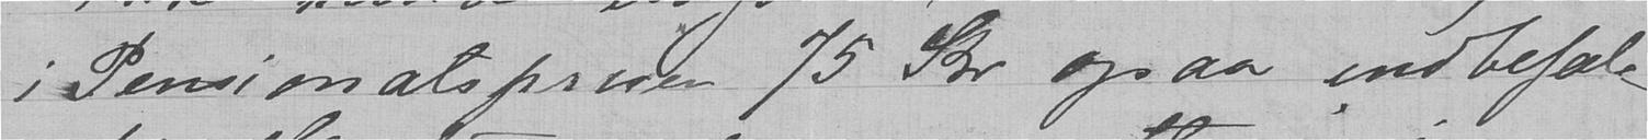i Pensionatsprisen 75 Kr ogsaa indbefat-
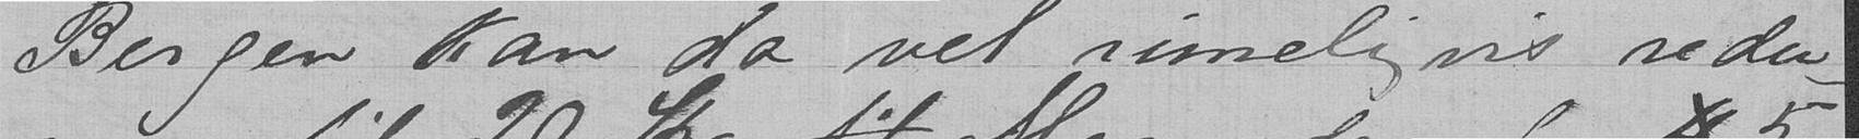Bergen kan da vel rimeligvis redu-
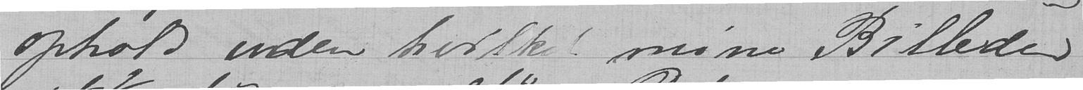ophold uden hvilket mine Billeder
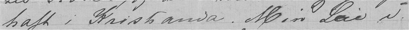haft i Kristiania. Min Leie i
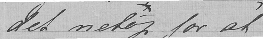det netop for at
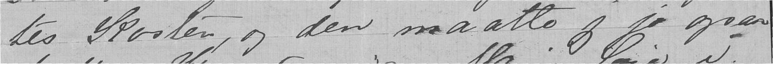tes Kosten, og den maatte jeg jo ogsaa
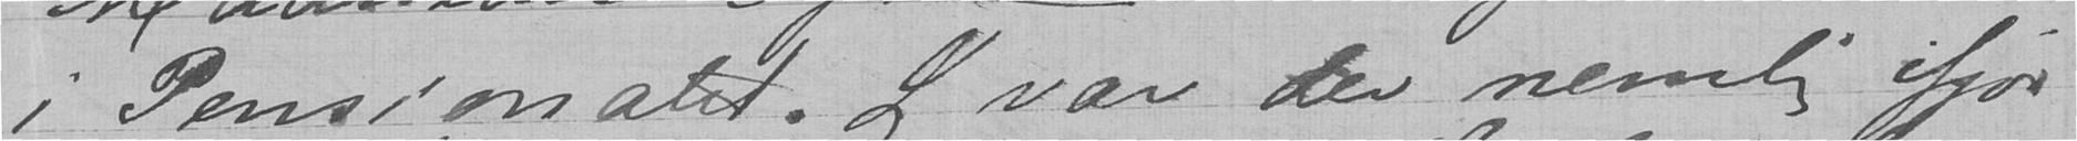i Pensionatet. Jeg var der nemlig ifjor
